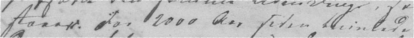staaer. For 2000 Aar siden tvivlede
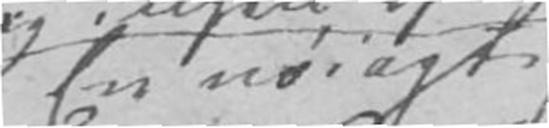En nøiagtig
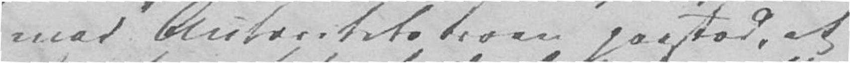mod Autoritetstroen paastod, at
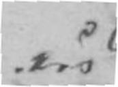nu
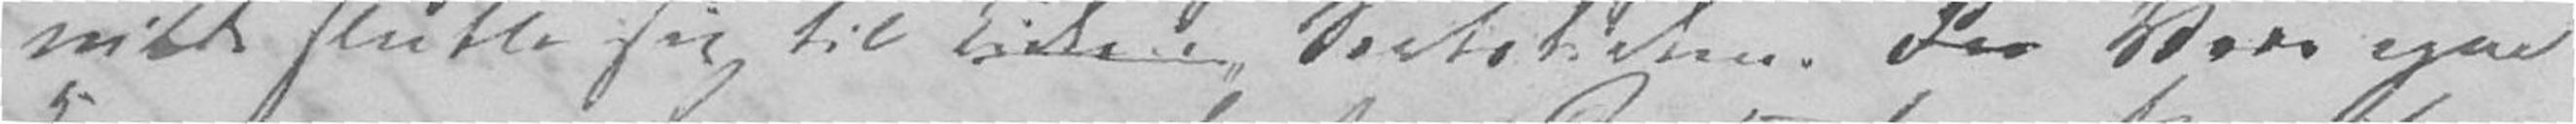vilde slutte sig til Sectskirken. Vore egne
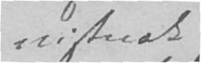vistnok
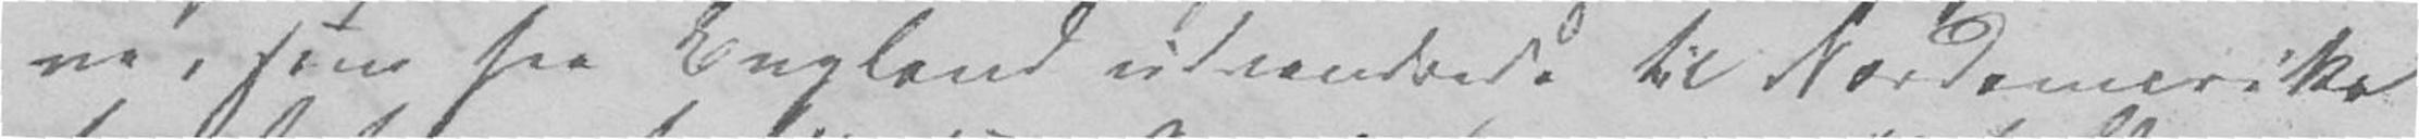ne, som fra England udvandrede til Nordamerika
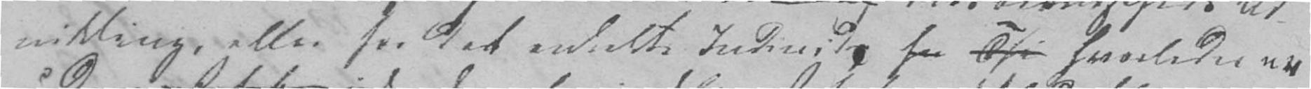vikling, eller for det enkelte Individ. Hvorledes er
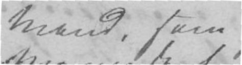Mand, som
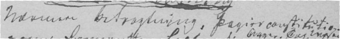Nærmere Betragtning, Papirconstitutio-
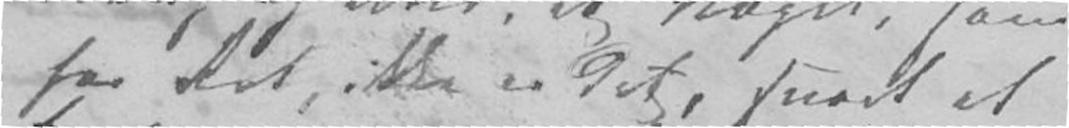for Ret, ikke er det, snart at
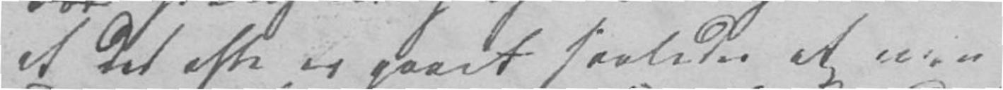at det ofte er gaaet saaledes at man
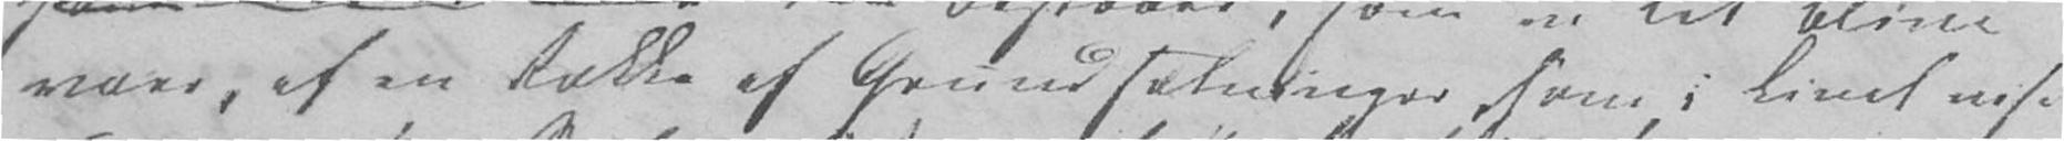vaer, af en Række af Grundsætninger, som i Livet vise
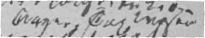Aager Dag kursen
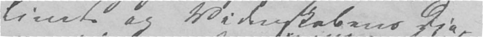Livets og Videnskabens Dia-
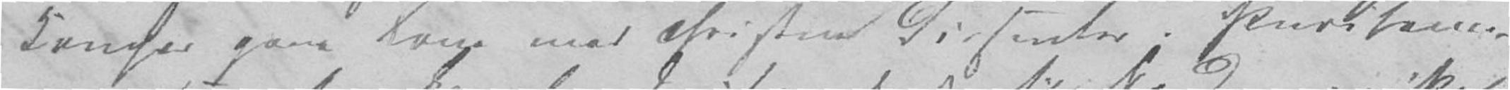Konger gaae Svige med christne Dissenter. Puritaner-
